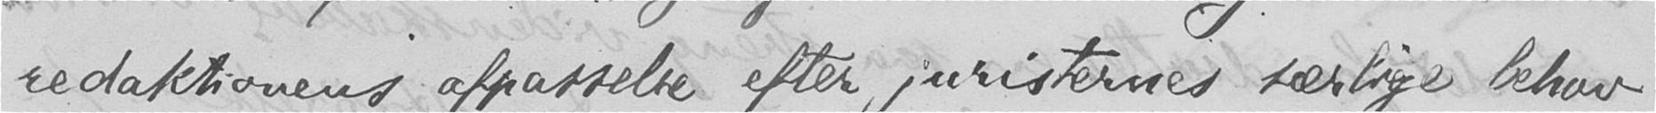redaktionens afpasselse efter juristernes særlige behov
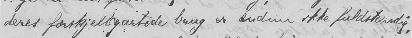deres forskjelligartede brug er endnu ikke fuldstændig,
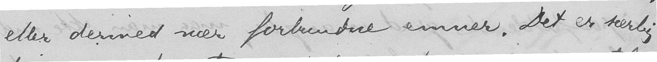eller dermed nær forbundne emner. Det er særlig
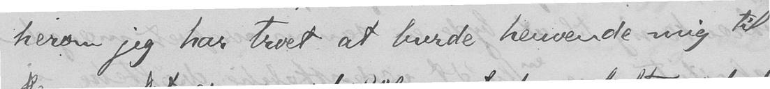herom jeg har troet at burde henvende mig til
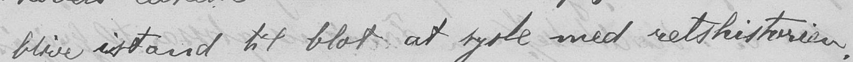blive istand til blot at sysle med retshistorien,
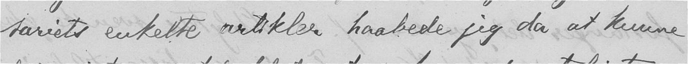sariets enkelte artikler haabede jeg da at kunne
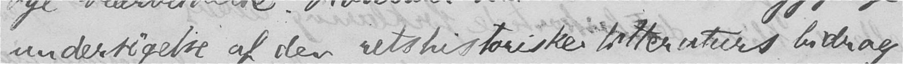undersøgelse af den retshistoriske litteraturs bidrag
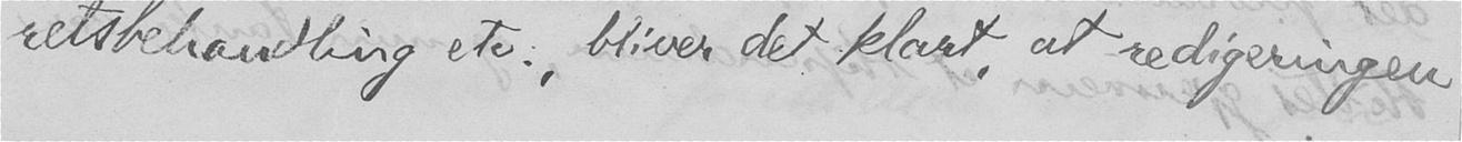retsbehandling etc., bliver det klart, at redigeringen
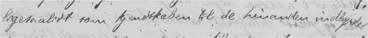ligesaalidt som kjendskaben til de hinanden indbyrdes
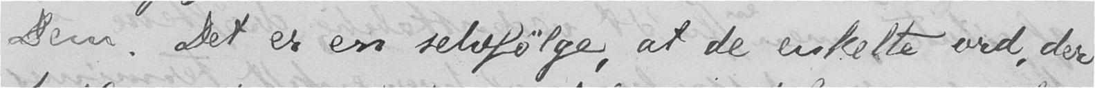Dem. Det er en selvfølge, at de enkelte ord der
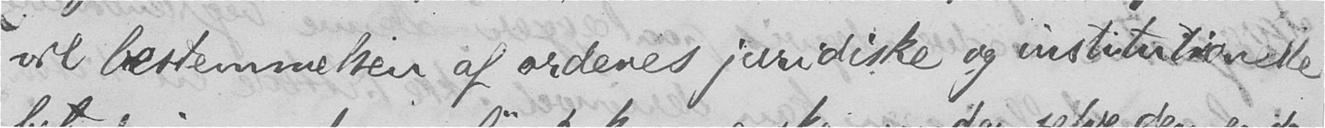vil bestemmelsen af ordenes juridiske og institutionelle
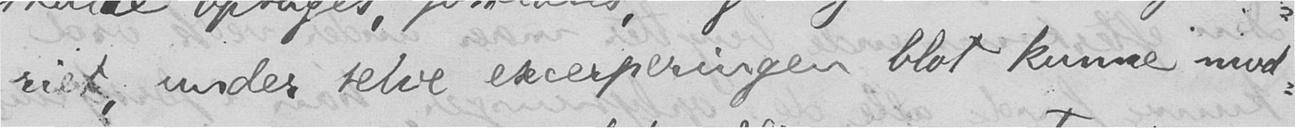riet, under selve excerperingen blot kunne mod-
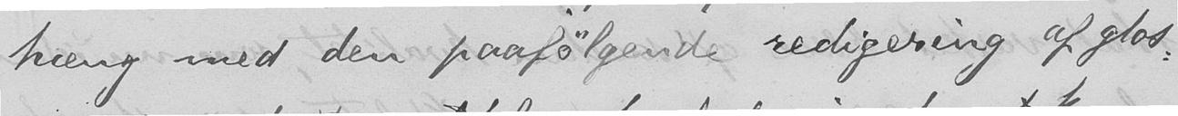hæng med den paafølgende redigering af glos-
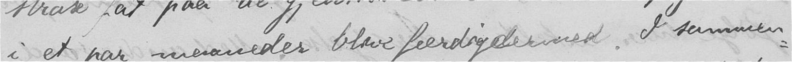i et par maaneder blive færdig dermed. I sammen-
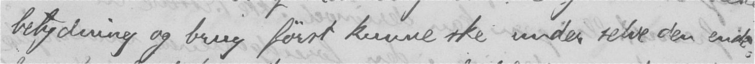betydning og brug først kunne ske under selve den ende-
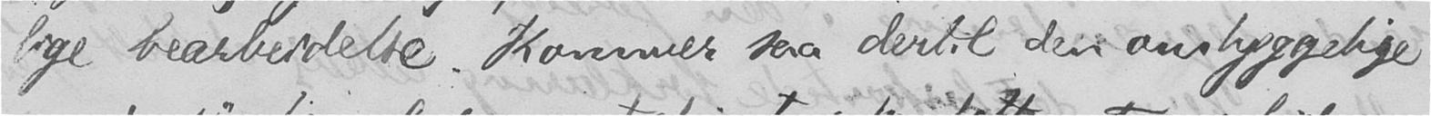lige bearbeidelse. Kommer saa dertil den omhyggelige
